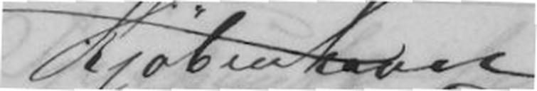Kjøbenhavn.
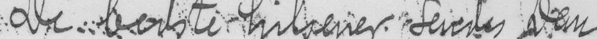De bedste hilsener sendes Dem
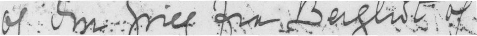og Fru Grieg fra Bergliot og.
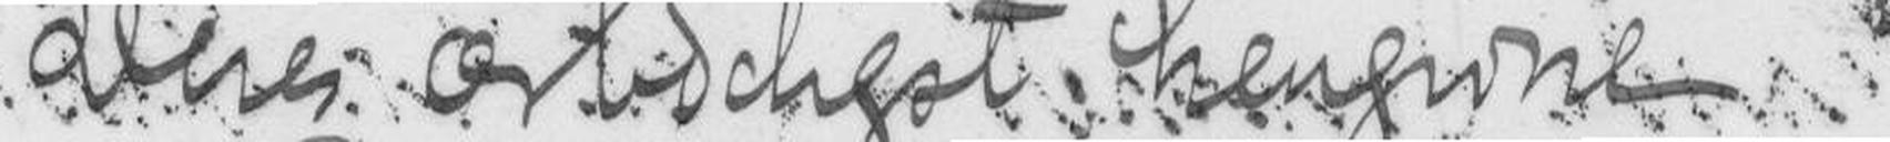Deres ærbødigst hengivne
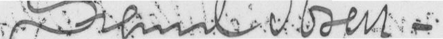Sigurd Ibsen
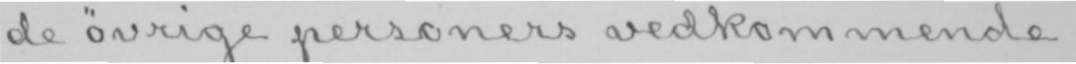de øvrige personers vedkommende.
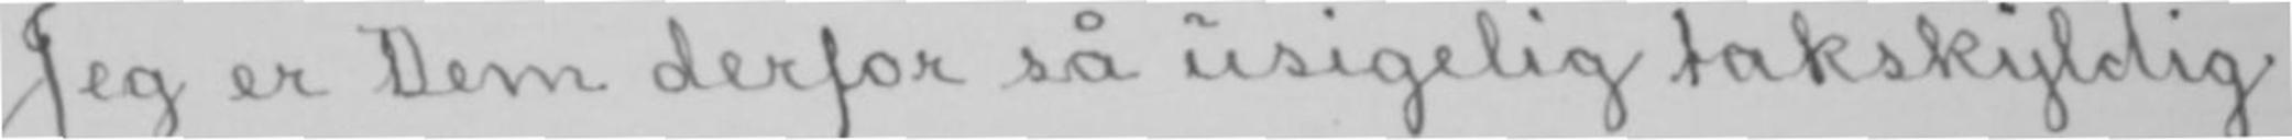Jeg er Dem derfor så usigelig takskyldig
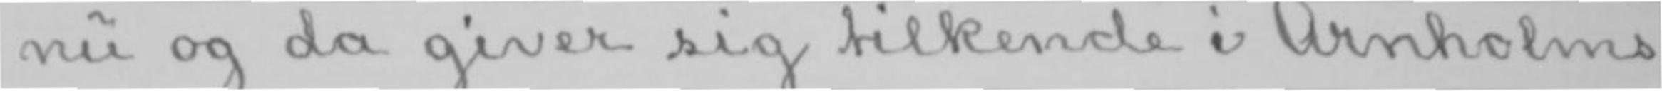nu og da giver sig tilkende i Arnholms
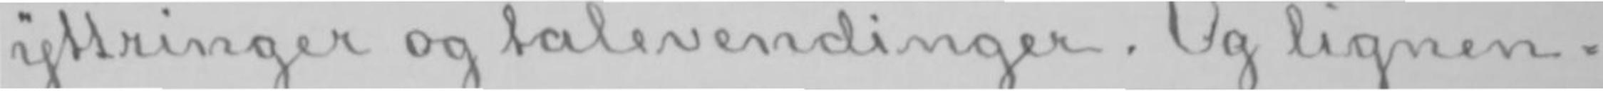yttringer og talevendinger. Og lignen-
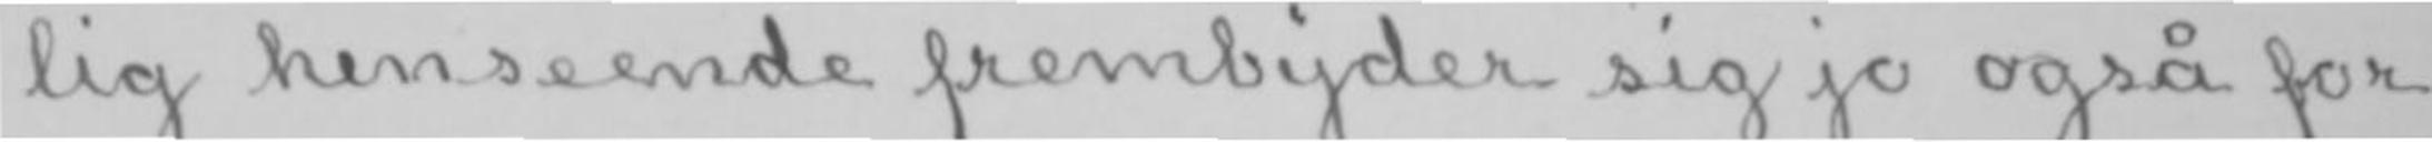lig henseende frembyder sig jo også for
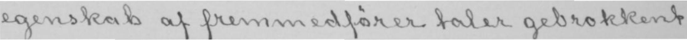egenskab af fremmedfører taler gebrokkent
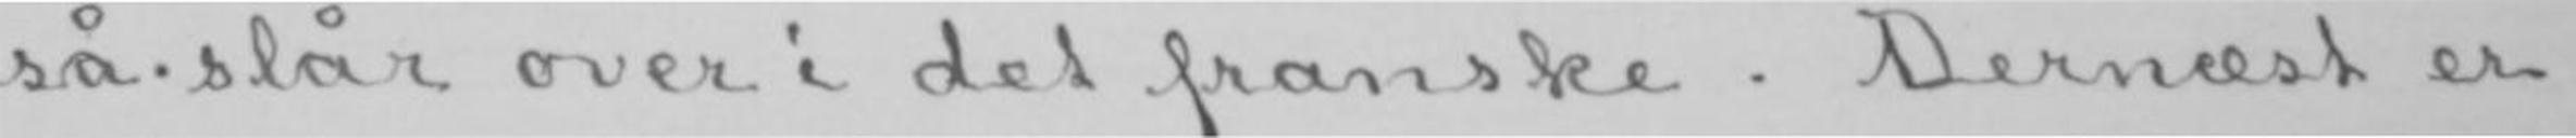så slår over i det franske. Dernæst er
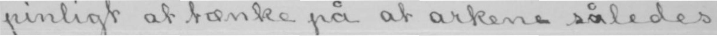pinligt at tænke på at arkene således
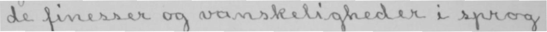de finesser og vanskeligheder i sprog-
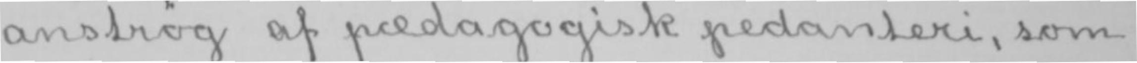anstrøg af pædagogisk pedanteri, som
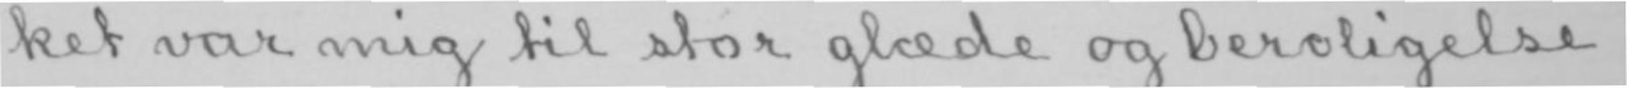ket var mig til stor glæde og beroligelse.
